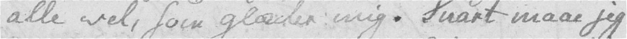alle vel, som glæder mig. Snart maae jeg
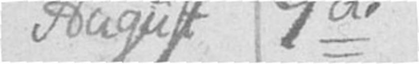August 7de
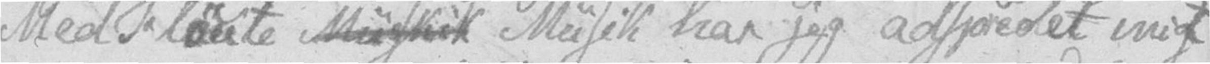Med Fløute Muskik Musik har jeg adspredet mit
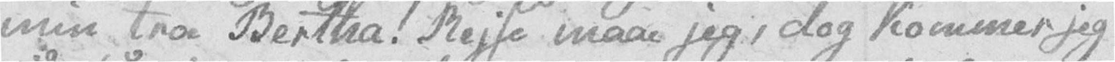min troe Bertha! Rejse maae jeg, dog kommer jeg
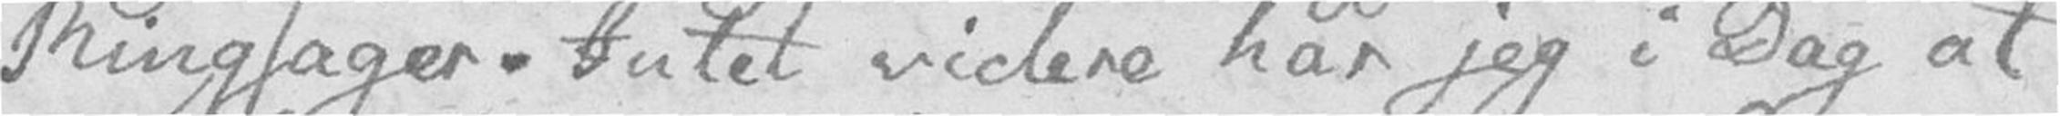Ringsager. Intet videre har jeg i Dag at
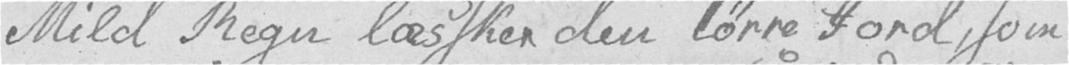Mild Regn læsker den tørre Jord, som
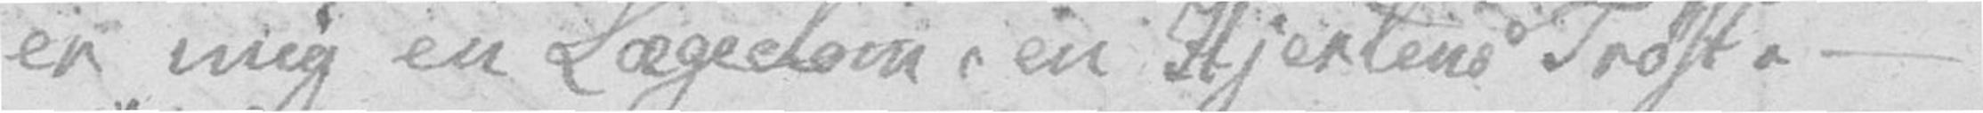er mig en Lægedom, en Hjertens Trøst. –
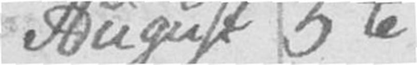August 5te
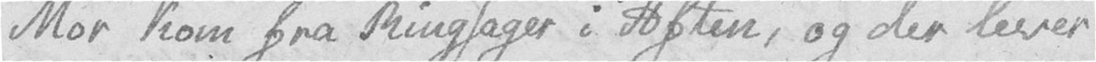Mor kom fra Ringsager i Aften, og der lever
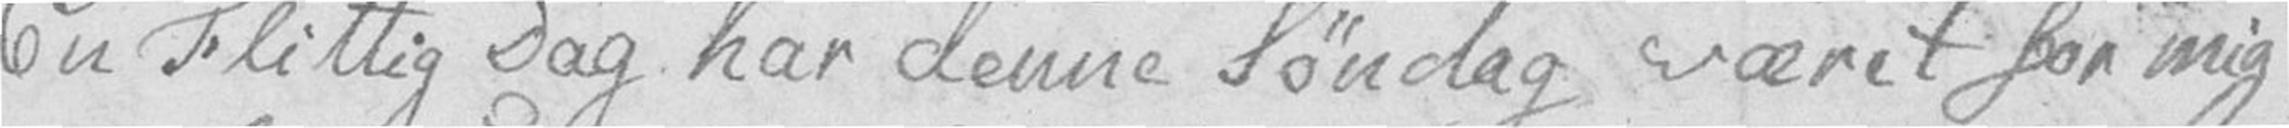En Flittig Dag har denne Søndag været for mig
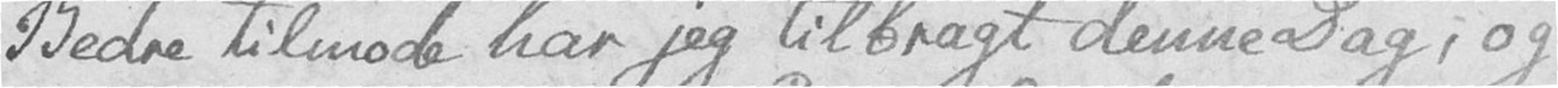Bedre tilmode har jeg tilbragt denne Dag, og
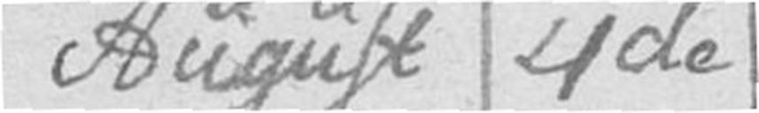August 4de
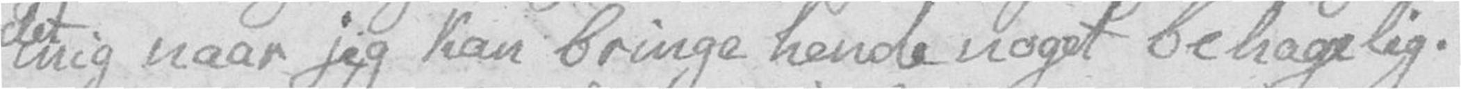det mig naar jeg kan bringe hende noget behagelig.
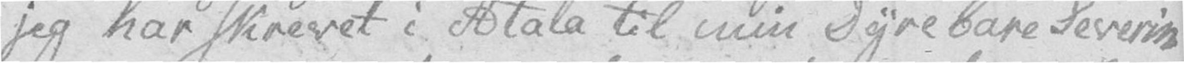jeg har skrevet i Atala til min Dyrebare Severine
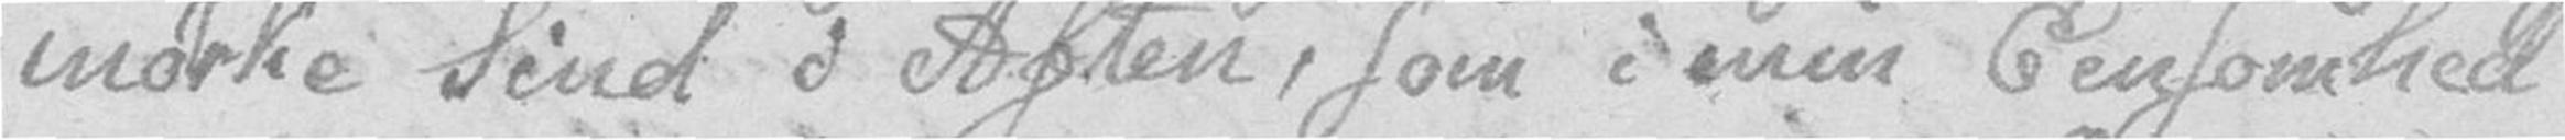mørke Sind i Aften, som i min Eensomhed
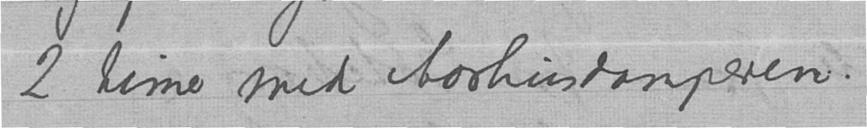2 timer med Aarhusdamperen.
